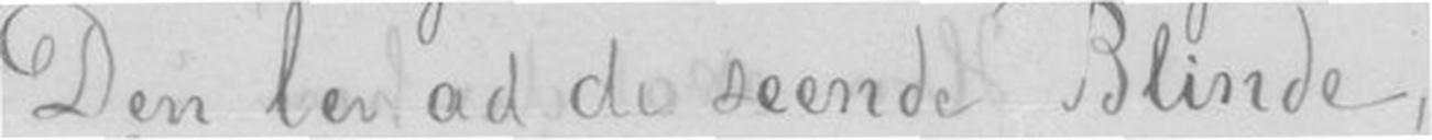Den ler ad de seende Blinde,
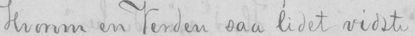Hvorom en Verden saa Lidet vidste
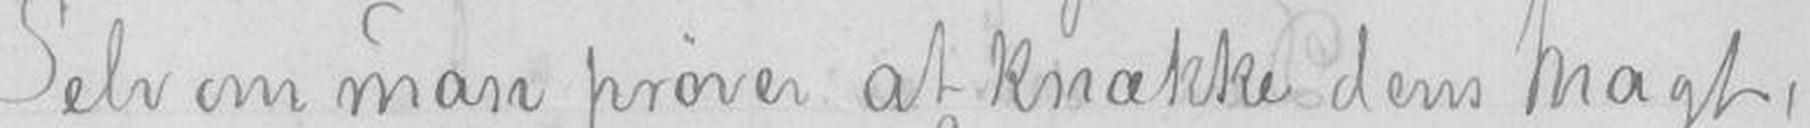Selv om man prøver at knække dens Magt,
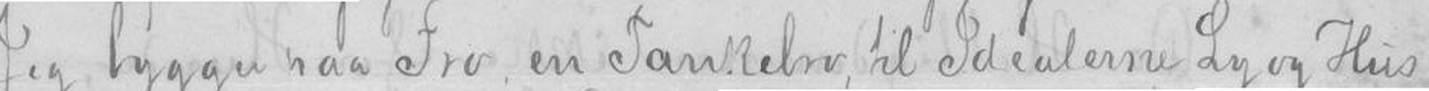Jeg bygger paa Tro en Tankebro, til Idealerne Ly og Hus
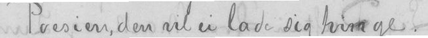Poesien, den vil ei lade sig tvinge
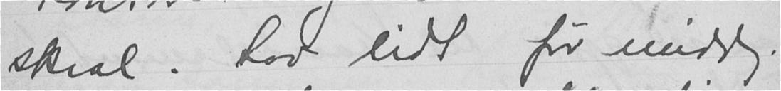skral. Sov lidt før middag.
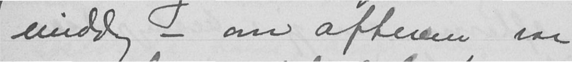middag - om aftenen var
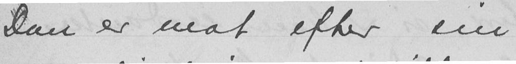Don er mat efter sin
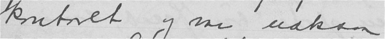kontoret og var noksaa
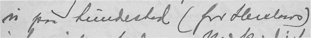vi paa Lundestad (for Herslows)
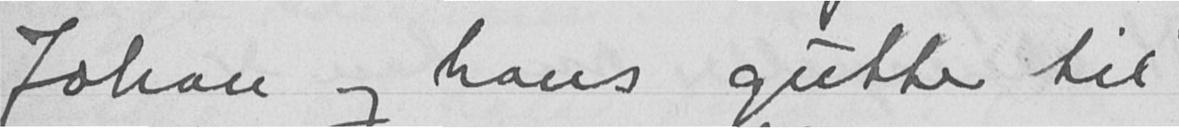Johan og hans gutter til
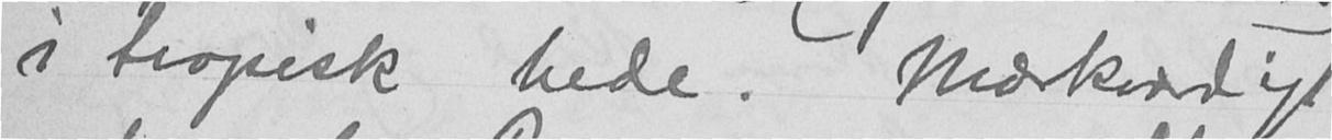i tropisk hede. Mærkverdigt
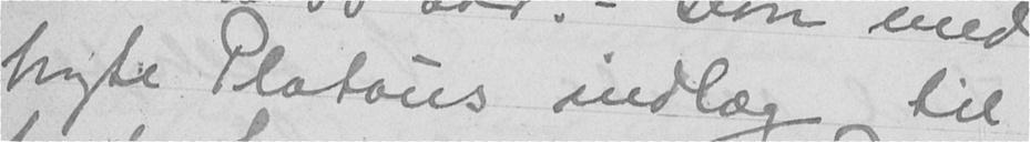bragte Platous indlæg til
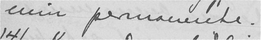min permanente.
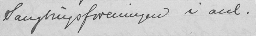Saugbrugsforeningen i anl.
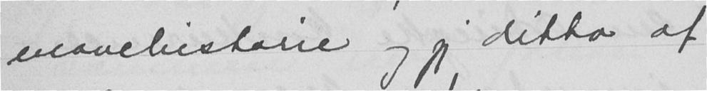mavehistorie og jeg ditto af
